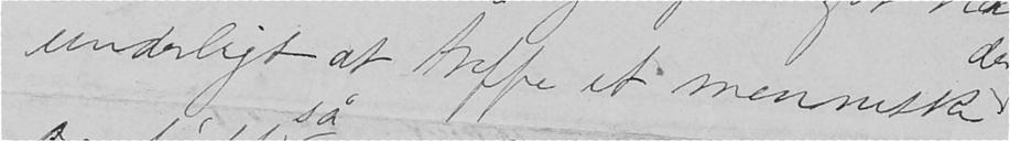underligt at treffe et menneske
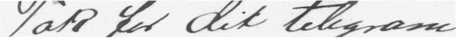Tak for dit telegram.
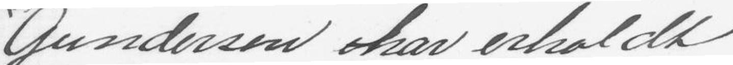Gundersen har erholdt
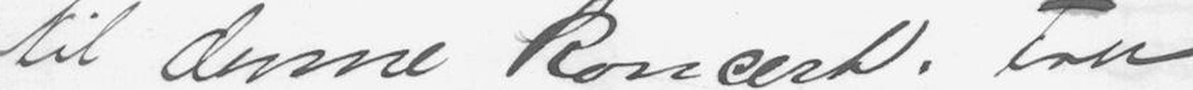til denne koncert. Fru
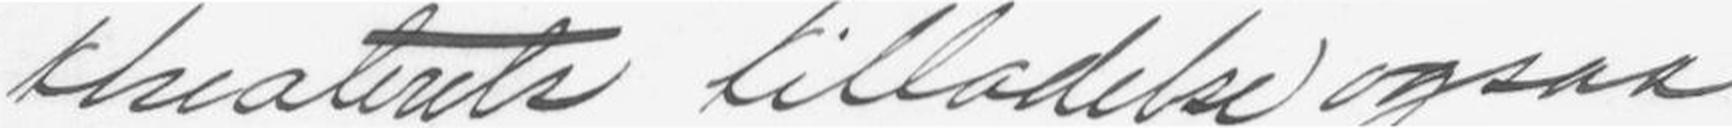theaterets tilladelse ogsaa
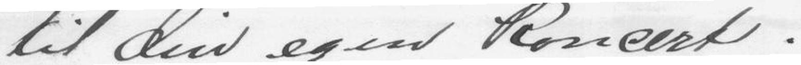til din egen koncert.
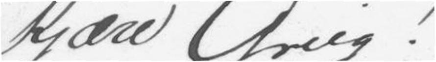Kjære Grieg!
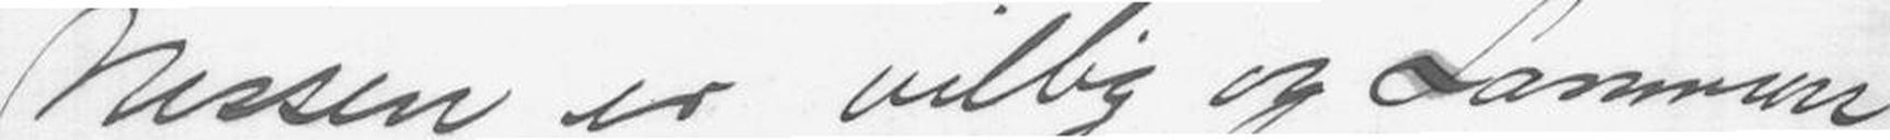Nissen er villig og Lammers
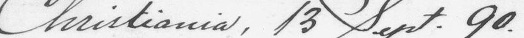Christiania, 13 Sept. 90.
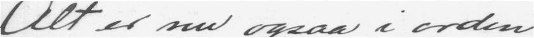Alt er nu ogsaa i orden
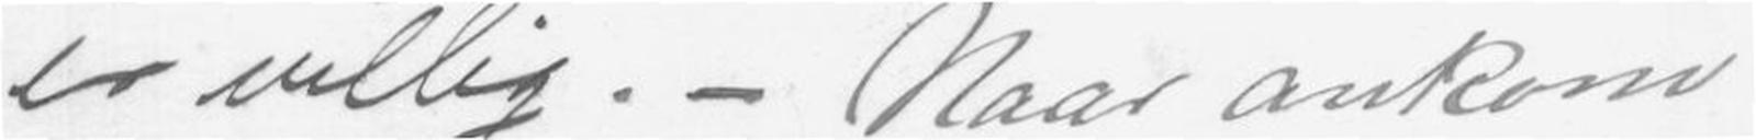er villig. - Naar ankom
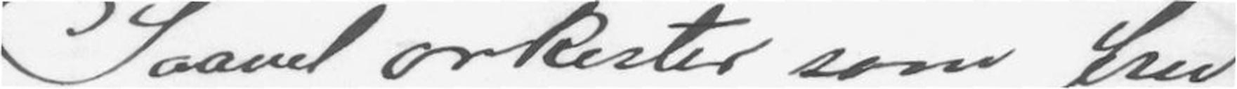Saavel orkester som fru
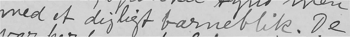med et dejligt barneblik. De
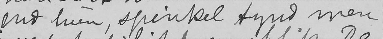end hun, spinkel tynd men
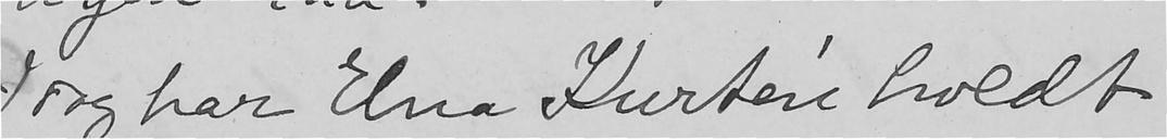Idag har Elna Kurtén holdt
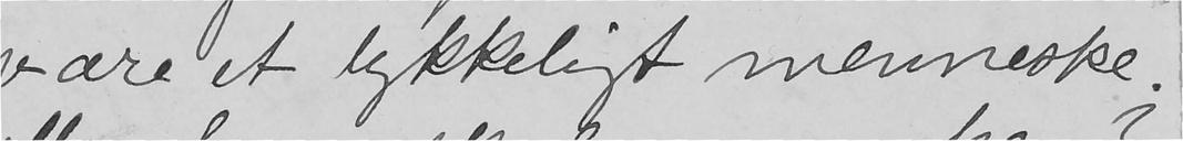være et lykkeligt menneske.
